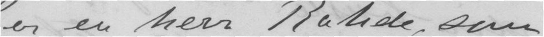er en herr Rahde, som
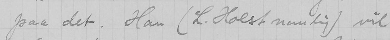paa det. Han (L. Holst nemlig) vil
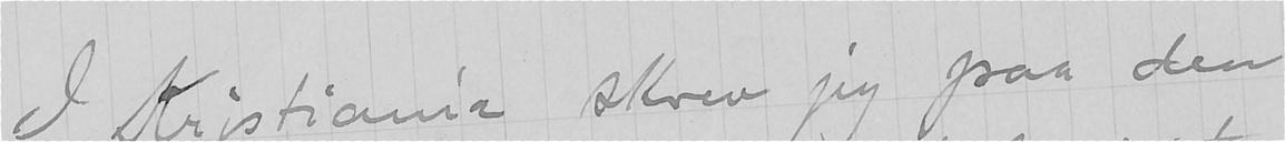I Kristiania skrev jeg paa den
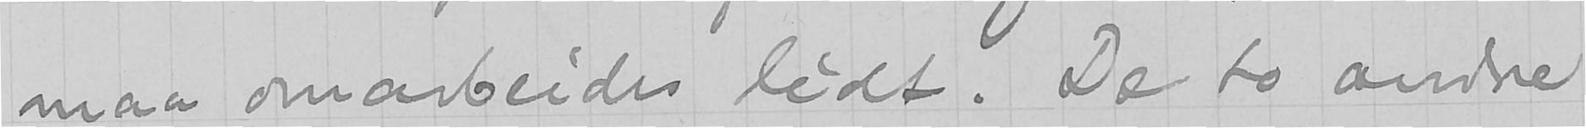maa omarbeides lidt. De to andre
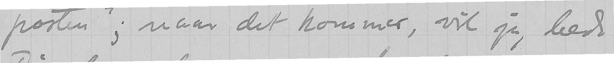posten"; naar det kommer, vil jeg bede
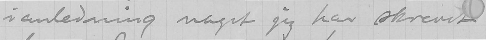i anledning noget jeg har skrevet
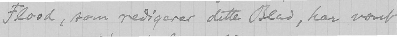Flood, som redigerer dette Blad, har været
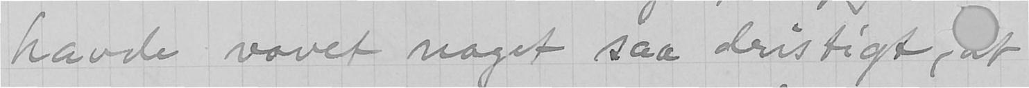havde vovet noget saa dristigt, at
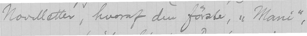Novelletter, hvoraf den første, "Mani",
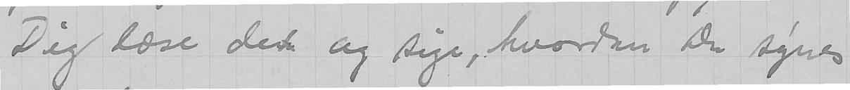Dig læse det og sige, hvordan De synes
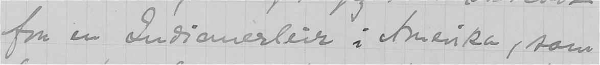fra en Indianerleir i Amerika, som
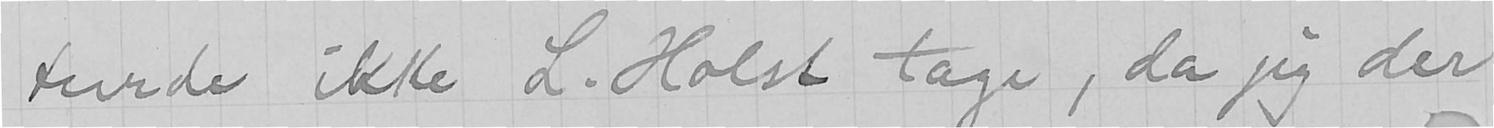turde ikke L. Holst tage, da jeg der
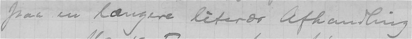paa en længere literær Afhandling
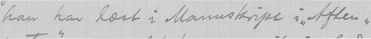han har læst i Manuskript i "Aften-
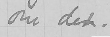om det.
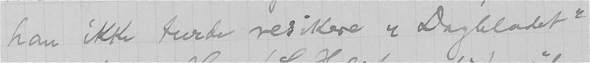han ikke turde resikere "Dagbladet"
